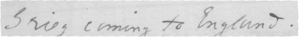Grieg coming to England?" -
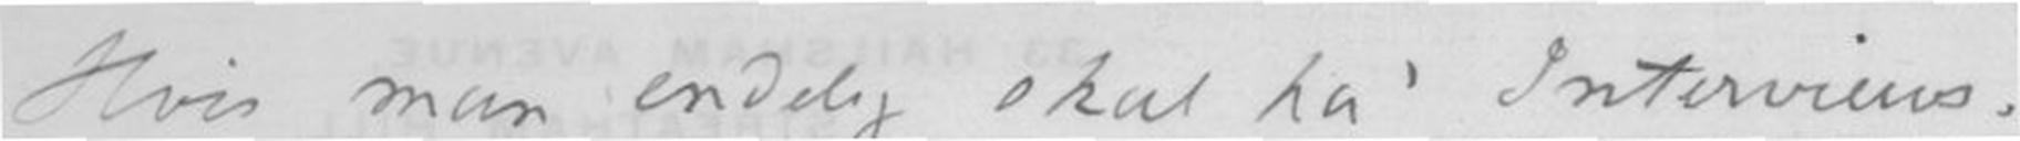Hvis man endelig skal ha interviews,
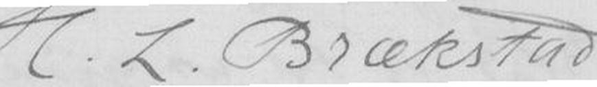H.L. Brækstad
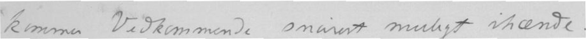kommer Vedkommende snarest muligt ihænde.
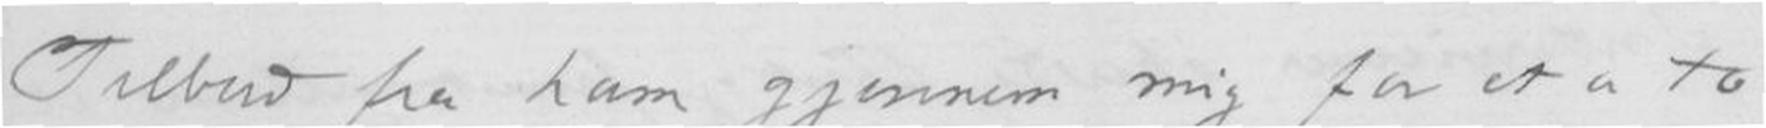Tilbud fra Ham gjennem mig for et a to
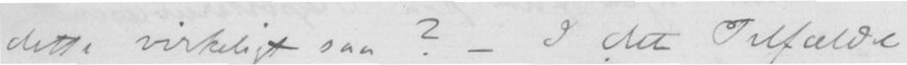dette virkelig saa? - I det Tilfælde,
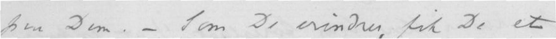paa Dem. - Som De erindrer, fik De et
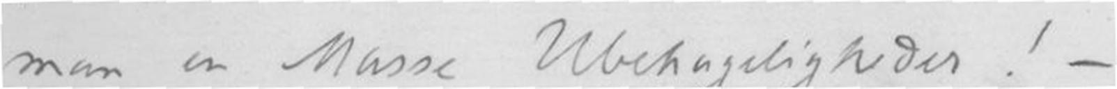man en Masse Ubehageligheder! -
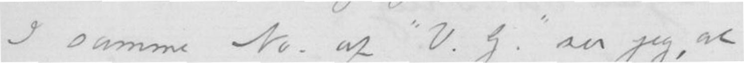I samme No. af "V.G." ser jeg, at
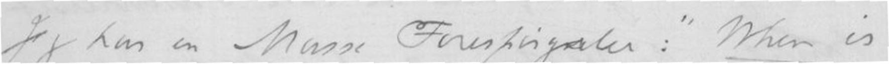Jeg har en Masse Forespørgsler: "When is
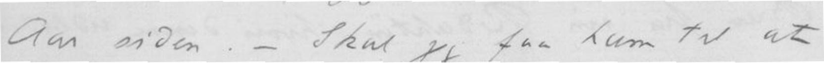Aar siden. - Skal jeg faa ham til at
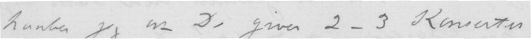haaber jeg at De giver 2-3 Konserter
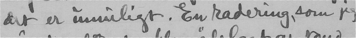det er umuligt. En radering, som jeg
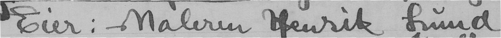Eier: Maleren Henrik Lundd
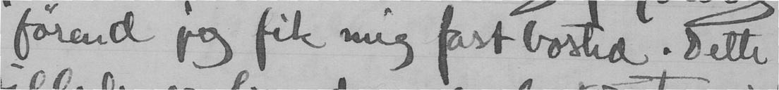førend jeg fik mig fast bosted. Dette
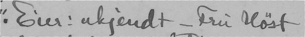Eier: ukjendt - Fru Høst
